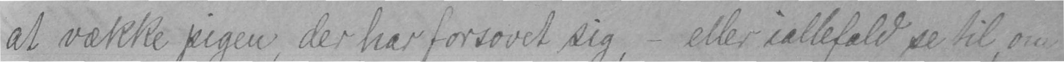at vække pigen, der har forsovet sig, - eller iallefald se til om
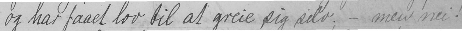og har faaet lov til at greie sig selv; - men nei!
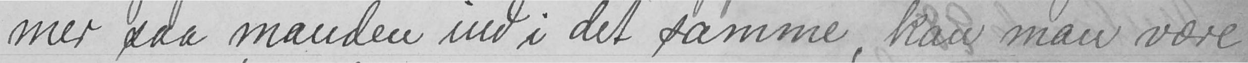mer saa manden ind i det samme, kan man være
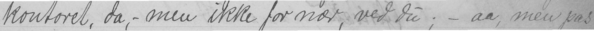kontoret, da, - men ikke for nær, ved du; - aa, men pas
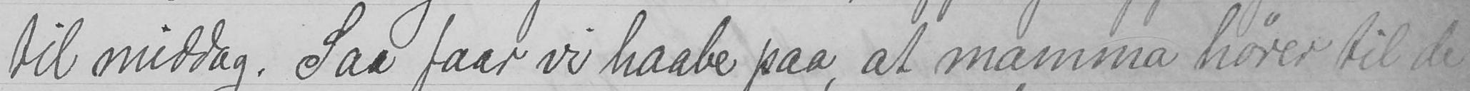til middag. Saa faar vi haabe paa, at mama hører til de
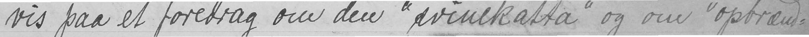vis paa et foredrag om den "svinekatta" og om "opbrænd-
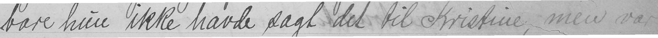bare hun ikke havde sagt det til Kristine, men var
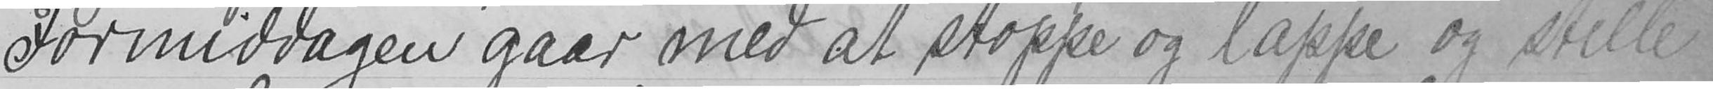Formiddagen gaar med at stoppe og lappe og stelle
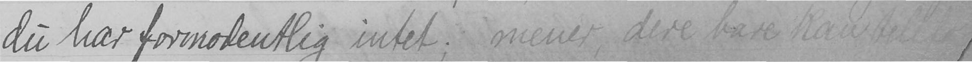du har formodentlig intet; mener, dere bare kan
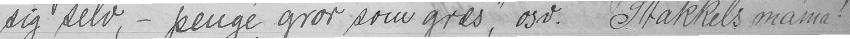sig selv, - penge gror som græs", osv. Stakkels mama!
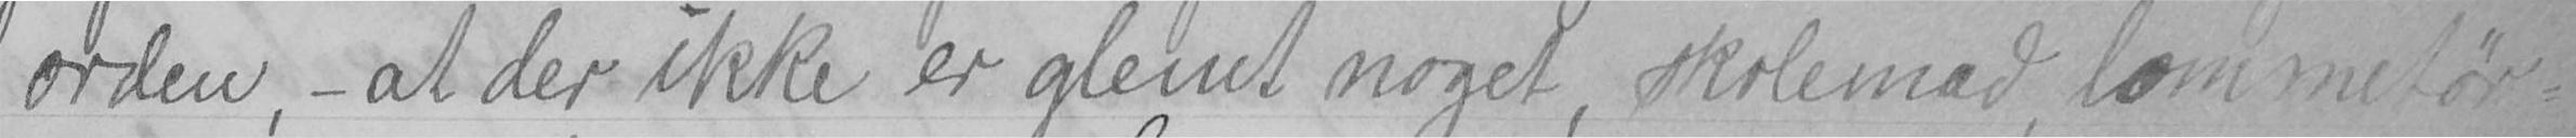orden, - at der ikke er glemt noget, skolemad, lommetør-
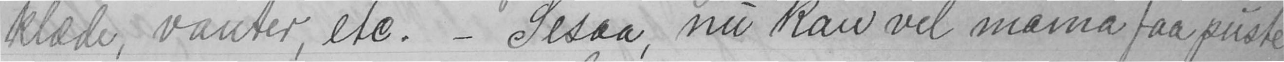klæde, vanter, etc. - Sesaa, nu kan vel mama faa puste
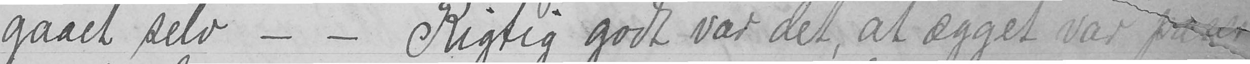gaaet selv - - Rigtig godt var det, at ægget var passe
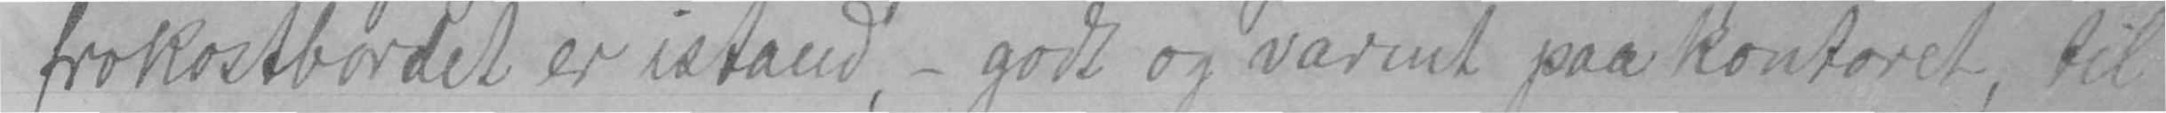frokostbordet er istand, - godt og varmt paa kontoret, til
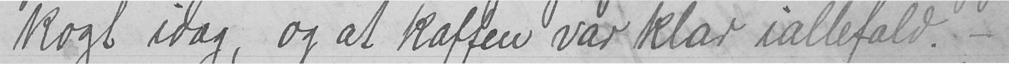kogt idag, og at kaffen var klar iallefald. -
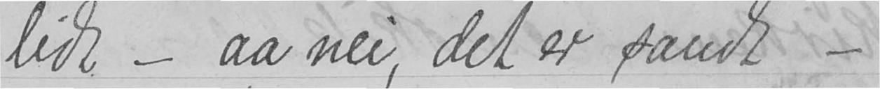lidt - aa nei, det er sandt -
